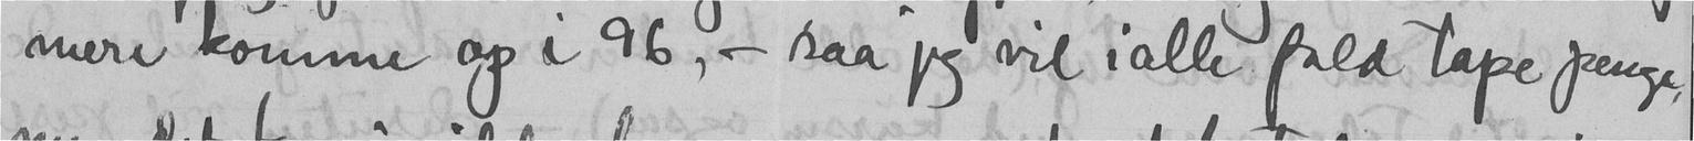mere komme op i 96, - saa jeg vil i alle fald tape penge,
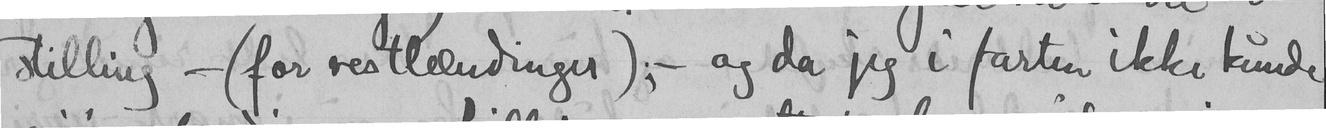stilling - (for vestlændinger); - da jeg i farten ikke kunde
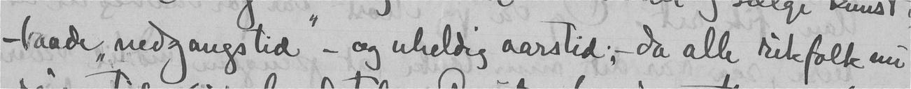- baade "nedgangstid" - og uheldig aarstid; - da alle rikfolk nu
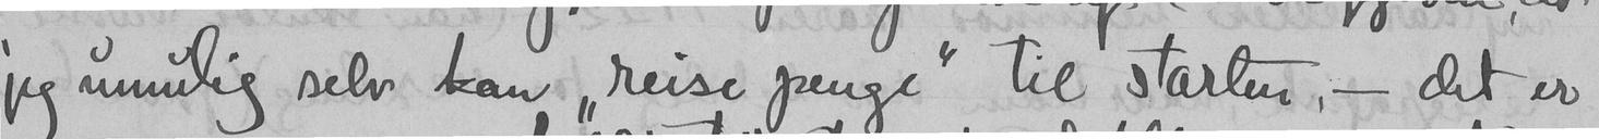jeg umulig selv kan "reise penge" til starten, - det er
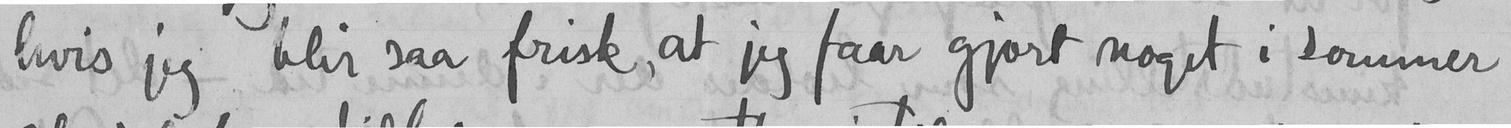hvis jeg blei saa frisk, at jeg faar gjort noget i sommer
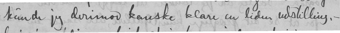kunde jeg derimod kanske klare en liden udstilling,-
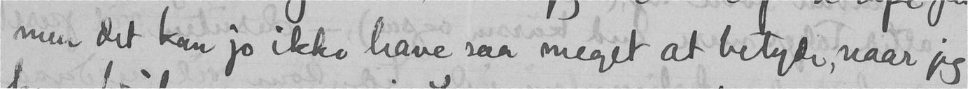men det kan jo ikke have saa meget at betyde, naar jeg
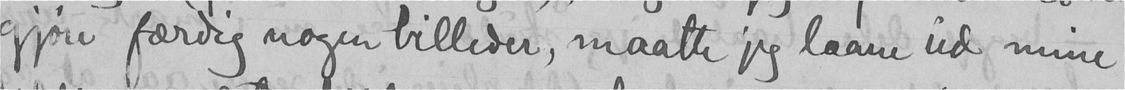gjøre færdig nogen billeder, maatte jeg laane ud mine
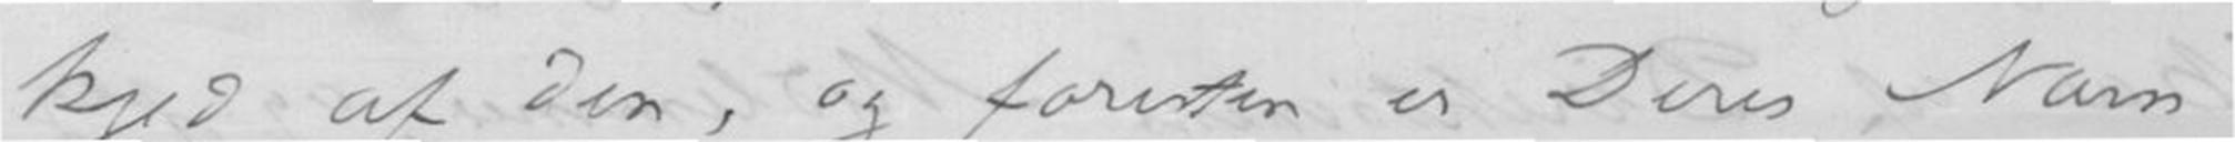kjed af den, og foresten er Deres Navn
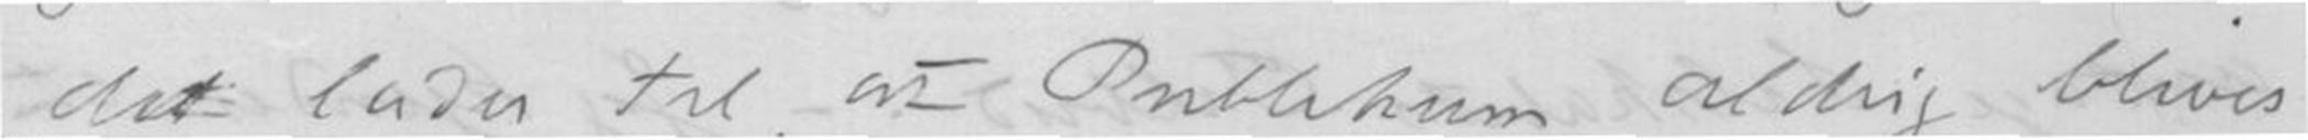det lader til, at Publikum aldrig bliver
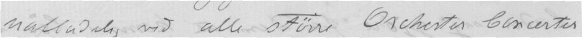uafladelig ved alle større Orchester Concerter
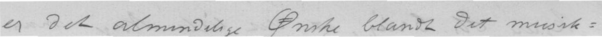er det almindelige Ønske blandt det musik-
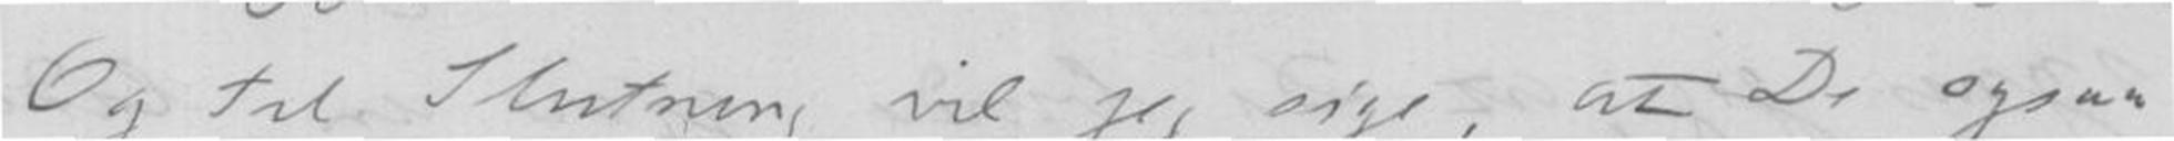Og til Slutning vil jeg sige, at De ogsaa
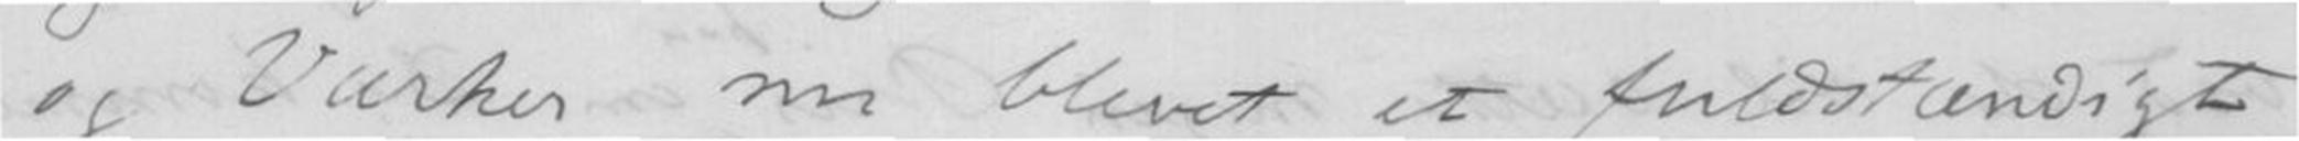og Værker nu blevet et fuldstændigt
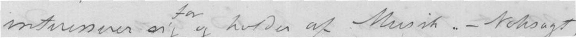interesserer sig og holder af Musik. - Nohsagt,
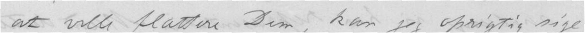at ville flattere Dem, kan jeg oprigtig sige
